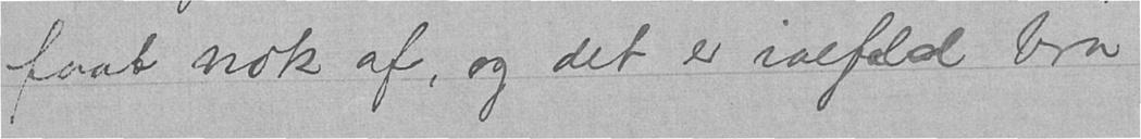faat nok af, og det er ialfald bra
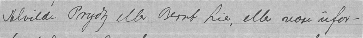Alvilde Prydz eller Bernt Lie, eller være ufor-
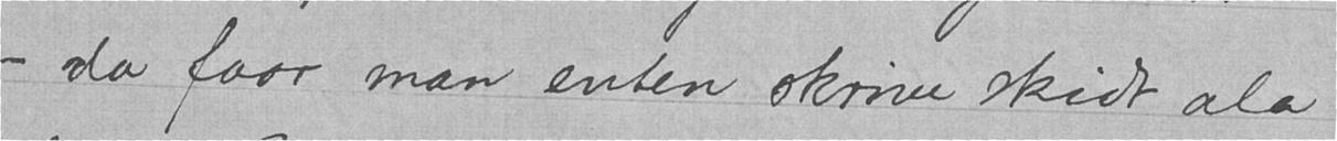- da faar man enten skrive skidt ala
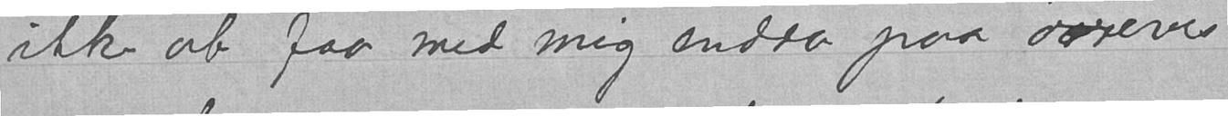ikke at faa med mig endda paa aarevis
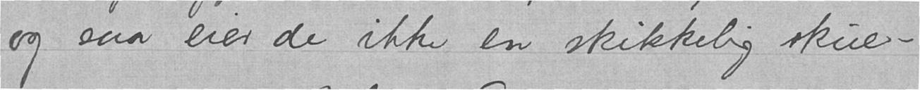og saa eier de ikke en skikkelig skue-
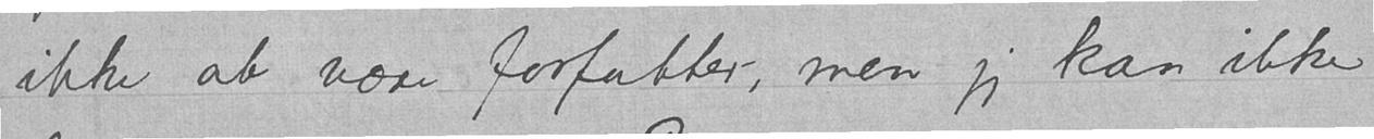ikke at være forfatter, men jeg kan ikke
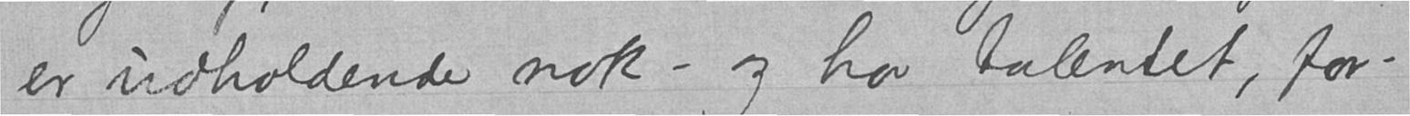er udholdende nok - og har talentet, for-
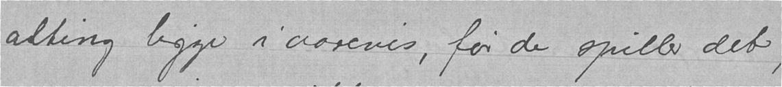alting ligge i aarevis, før de spiller det,
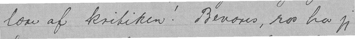lære af kritiken! Bevares, ros har jeg
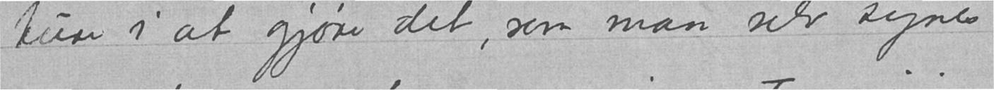ture i at gjøre det, som man selv synes
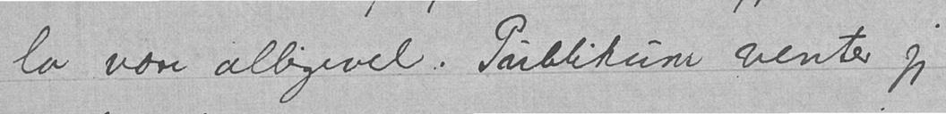la være alligevel. Publikum venter jeg
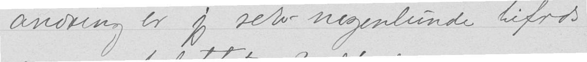andring er jeg selv nogenlunde tifreds
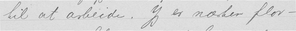til at arbeide. Jeg er næsten flov -
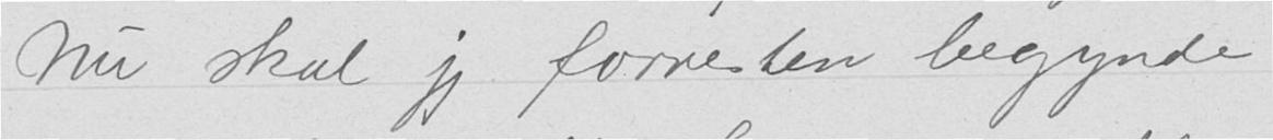Nu skal jeg forresten begynde
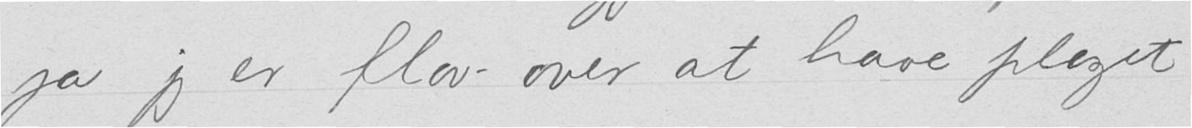ja jeg er flov over at have plaget
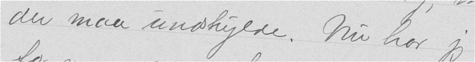der maa undskylde. Nu har jeg
